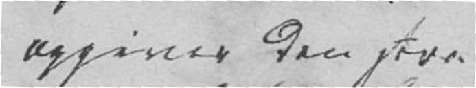opgiver den store
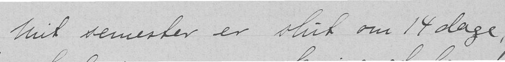Mit semester er slut om 14 dage,
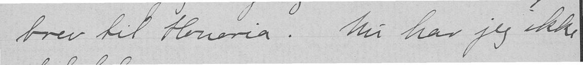brev til Honoria. Nu har jeg ikke
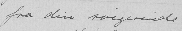fra din svigerinde
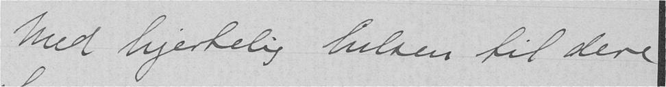Med hjertelig hilsen til dere
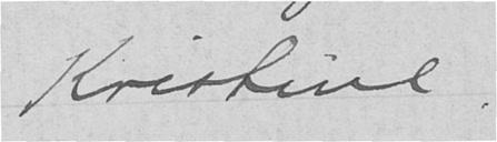Kristine.
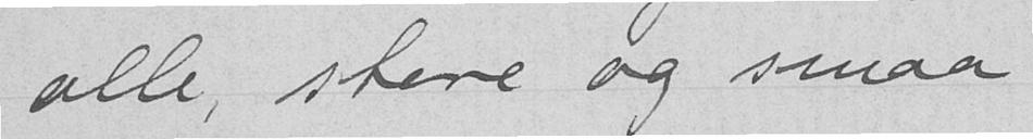alle, store og smaa
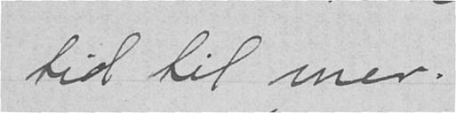tid til mer.
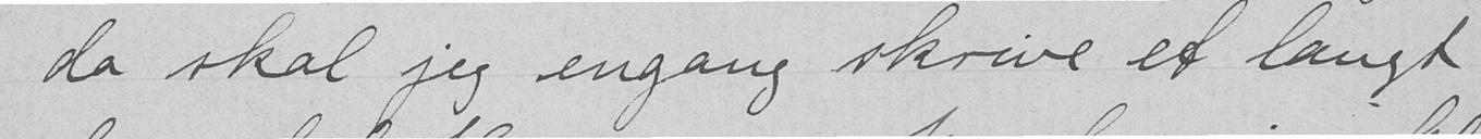da skal jeg engang skrive et langt
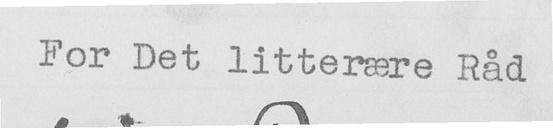For Det litterære Råd
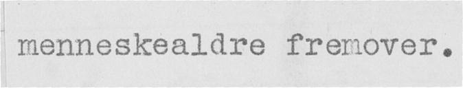menneskealdre fremover.
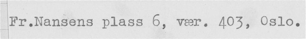Fr.Nansens plass 6, vær. 403, Oslo.
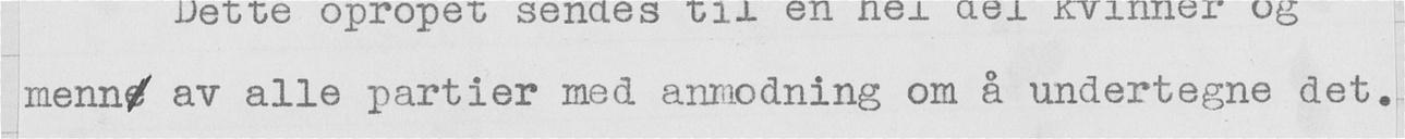menne av alle partier med anmodning om å undertegne det.
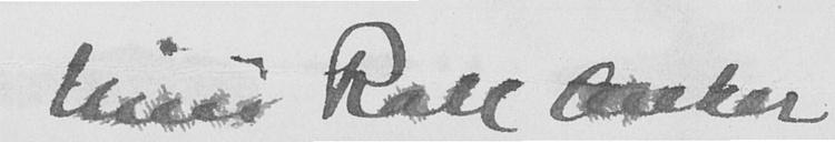Nini Roll Anker
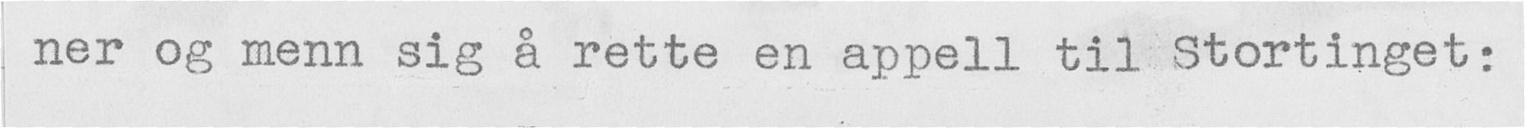ner og menn sig å rette en appell til Stortinget:
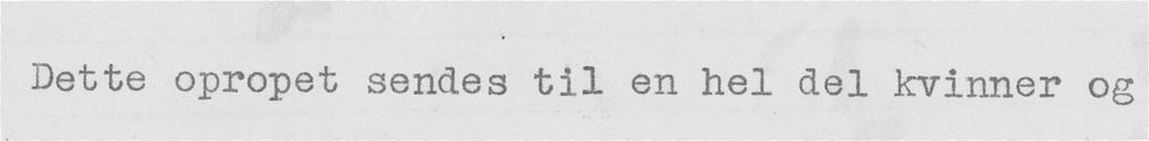Dette opropet sendes til en hel del kvinner og
